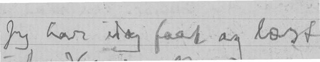Jeg har idag faat og læst
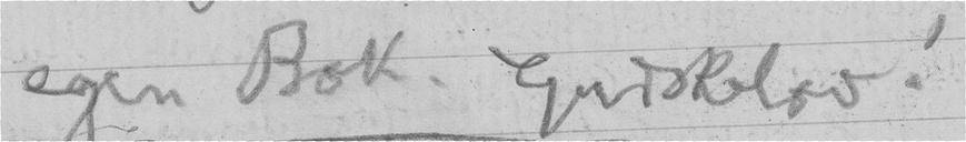egen Bok. Gudskelov!
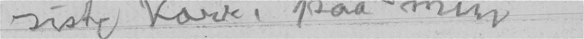siste Korr. paa min
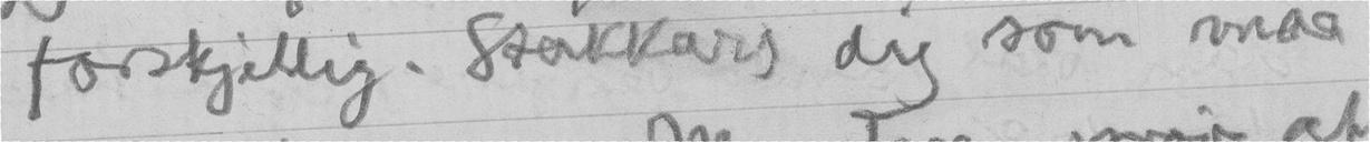forskjellig. Stakkars dig som maa
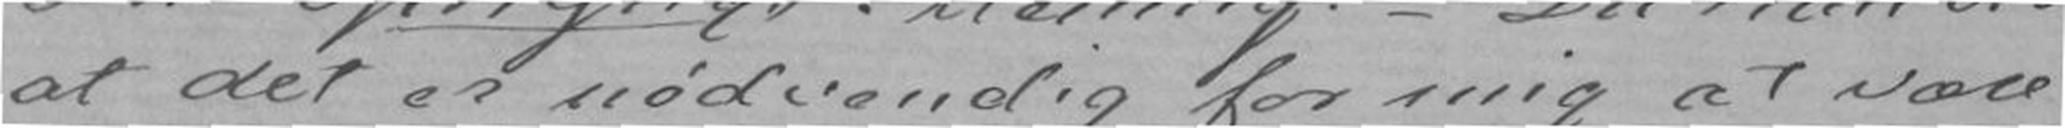at det er nødvendig for mig at være
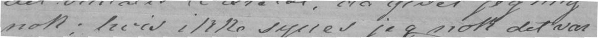nok; hvis ikke synes jeg nok det var
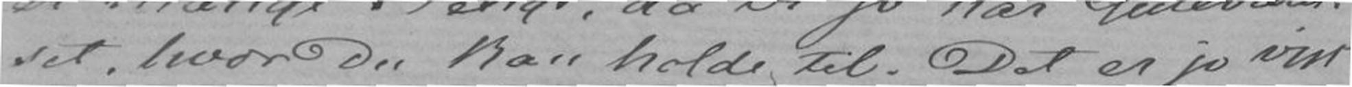set, hvor Du kan holde til. Det er jo vist
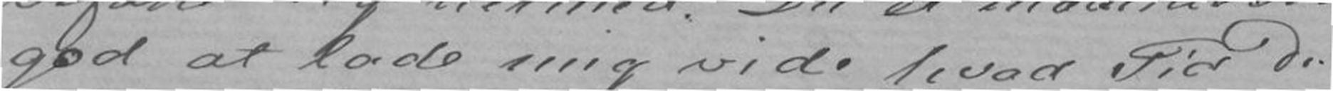god at lade mig vide hvad Tid Du
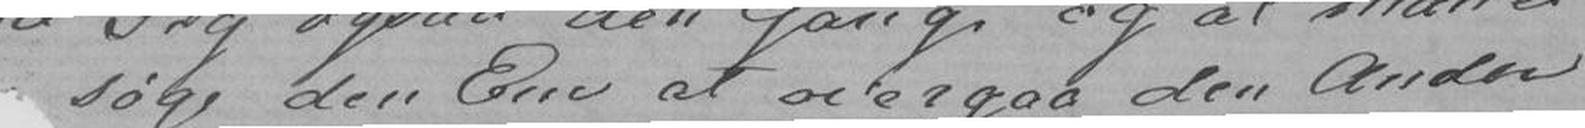søge den Ene at overgaa den Andre
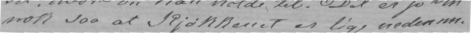nok saa at Kjøkkenet er lige nedenun-
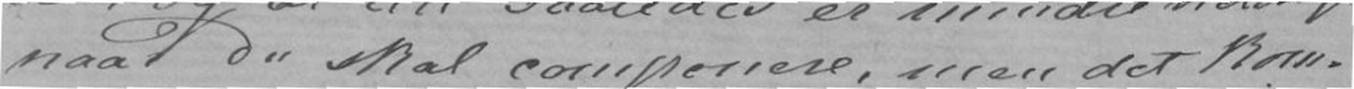naar Du skal componere, men det kom-
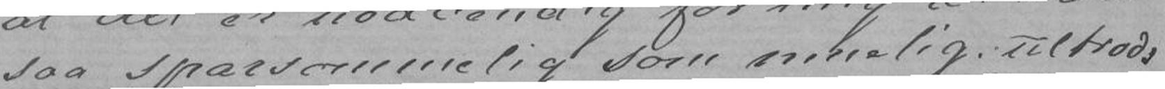saa sparsommelig som muelig tiltrods
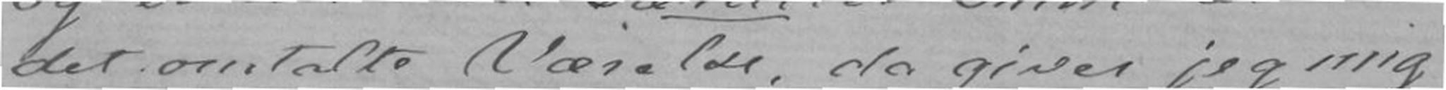det omtalte Værelse, da giver jeg mig
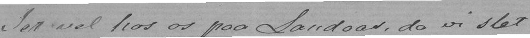Jer vel hos os paa Landaas, da vi slet
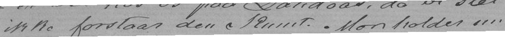ikke forstaar den Knut. Mor holder nu
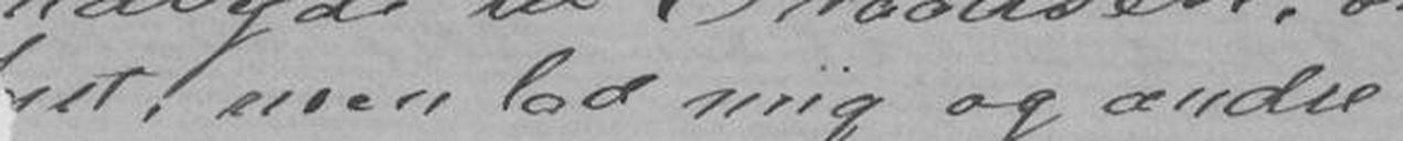st, men lod mig og andre
